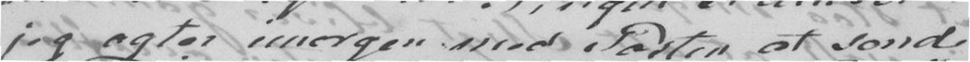jeg agter imorgen med Posten at sende
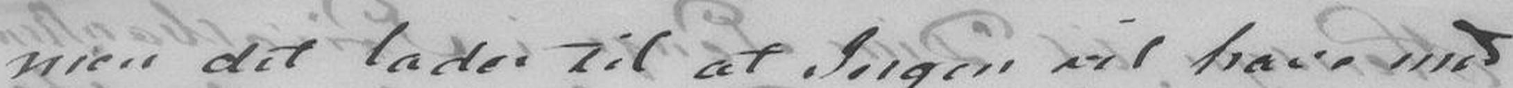men det lader til at Ingen vil have med
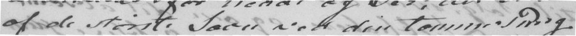af de største Savn ved din tomme Pung.
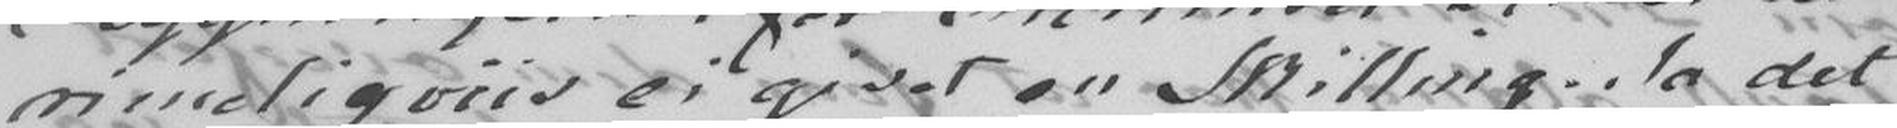rimeligviis ei givet en Skilling. Ja det
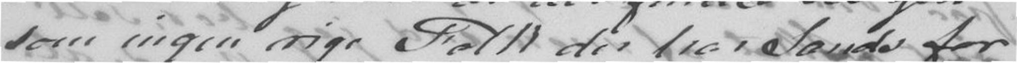som ingen rige Folk der har Sands for
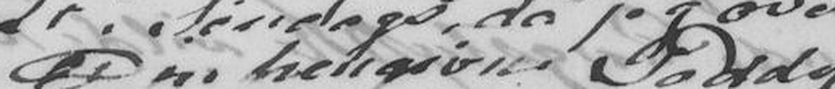Din hengivne Paddy.
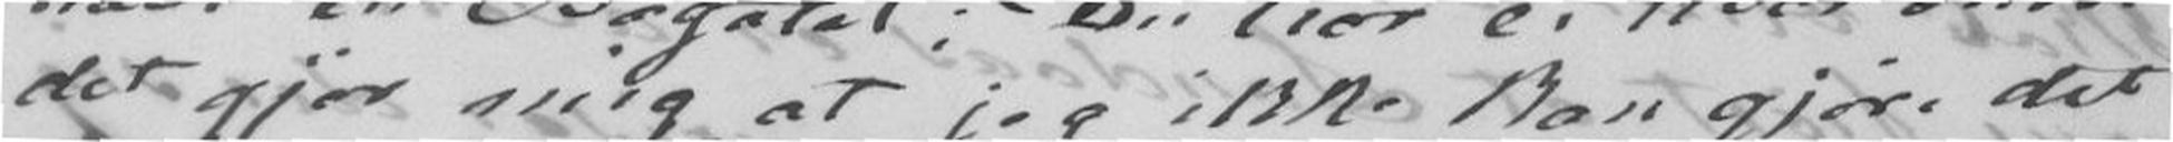det gjør mig at jeg ikke kan gjøre det
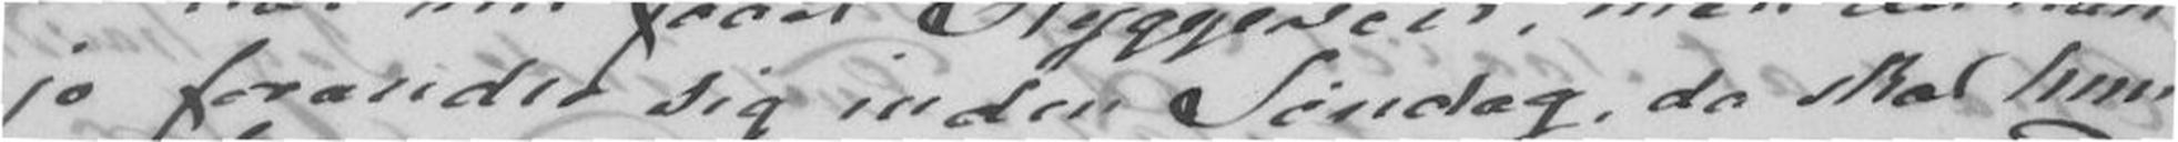jo forandre sig inden Søndag, da skal hun
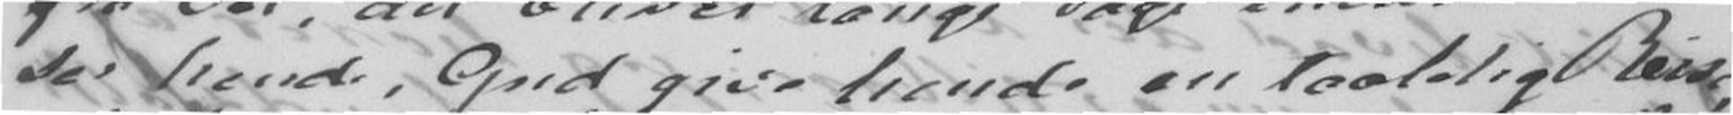ser hende, Gud give hende en taalelig Reise,
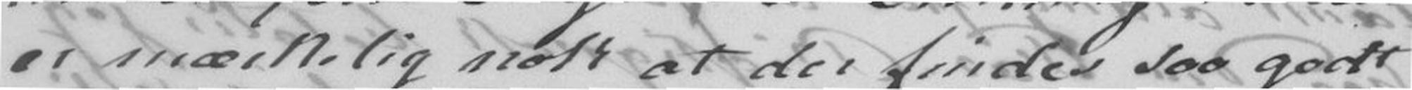er mærkelig nok at der findes saa godt
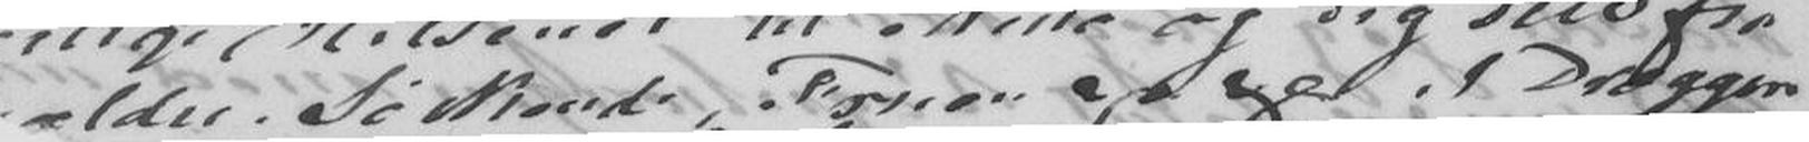ældre, Søskende, Fruen ogc ogc. I Dræggen
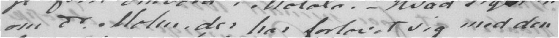om Dr Mohn, der har forlovet sig med den
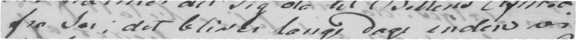fra Jer; det bliver lange Dage inden vi
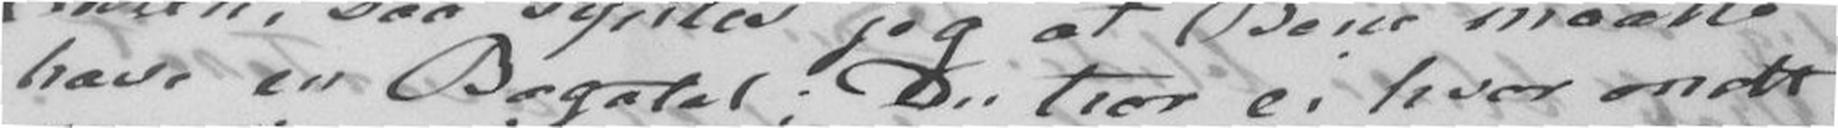have en Bagatel; Du tror ei hvor ondt
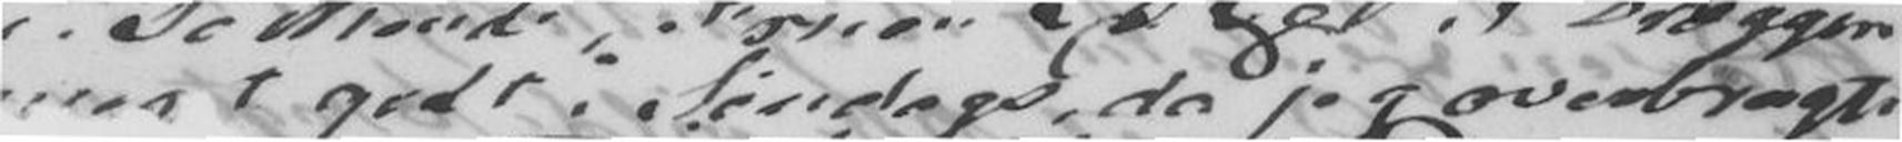t godt i Søndags, de jeg overbragte
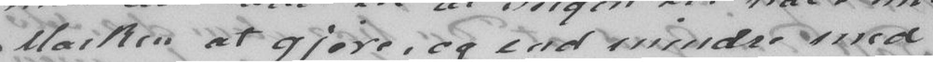Marken at gjøre, og end mindre med
